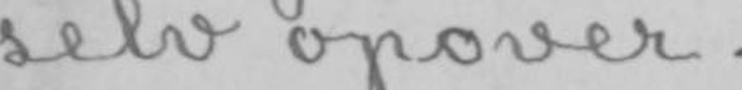selv opover.
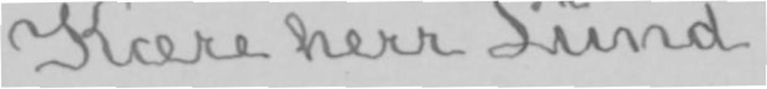Kære herr Lund,
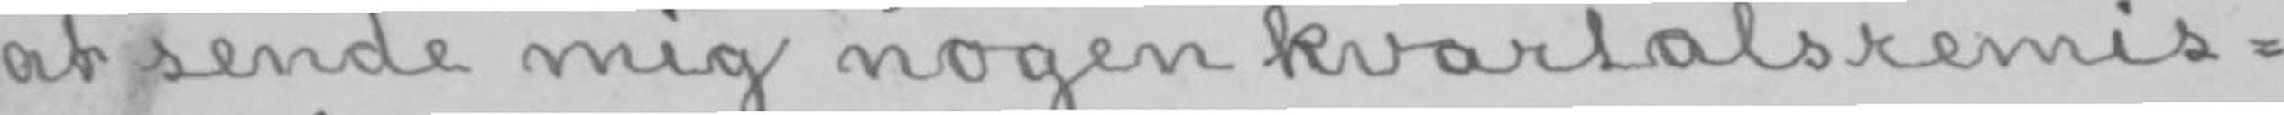at sende mig nogen kvartalsremis-
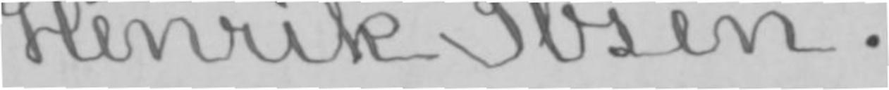Henrik Ibsen.
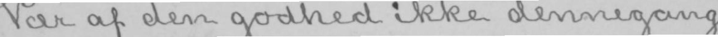Vær af den godhed ikke dennegang
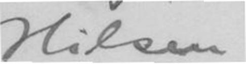Hilsen!
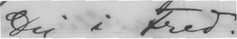Dig i Fred!
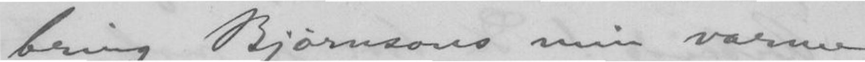bring Bjørnsons min varme
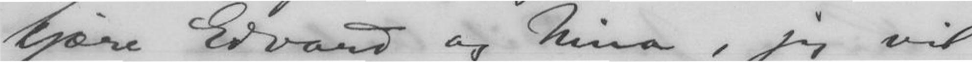kjære Edvard og Nina, jeg vil
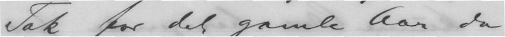Tak for det gamle Aar da,
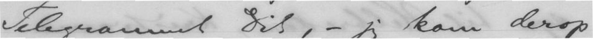Telegrammet Dit, - jeg kom derop
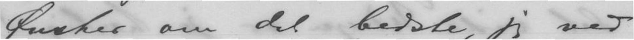Ønsker om det bedste, jeg ved,
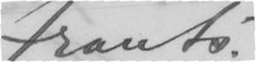Frants.
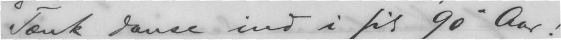Tænk danse ind i sit 90de Aar!
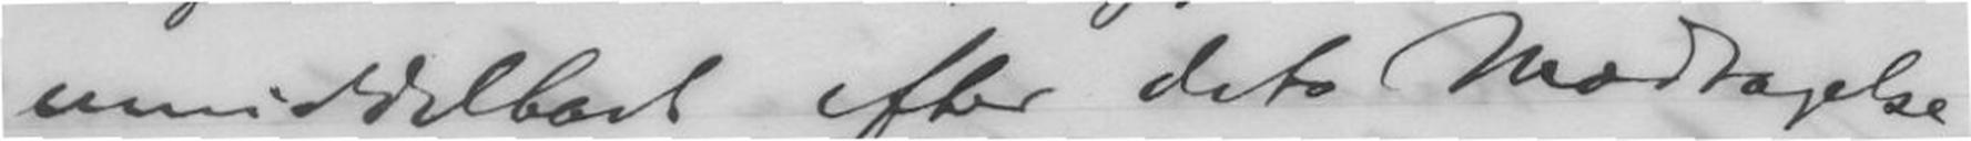umiddelbart efter dets Modtagelse,
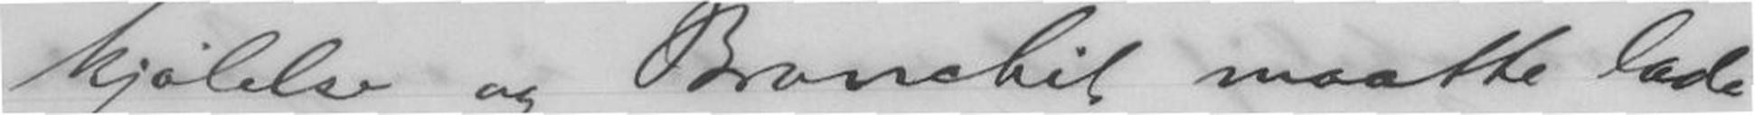kjølelse og Bronchit maatte lade
